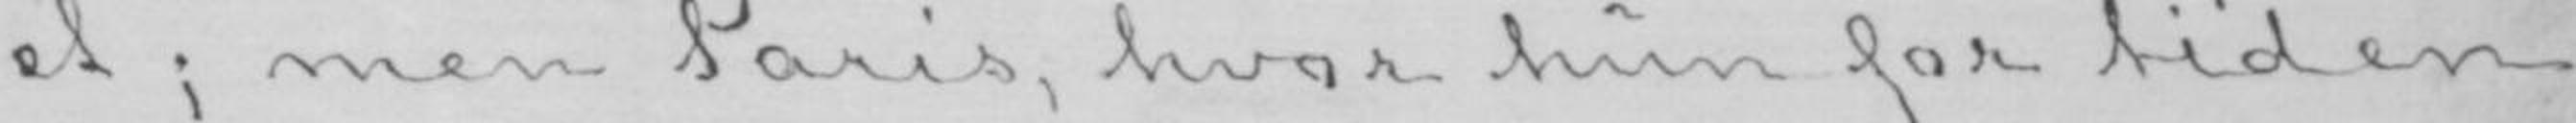et; men Paris, hvor hun for tiden
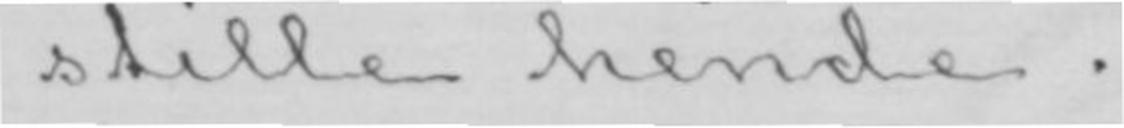stille hende.
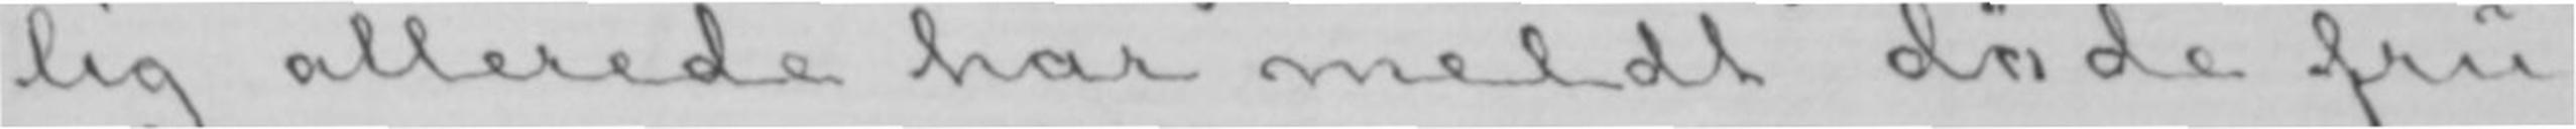lig allerede har meldt døde fru
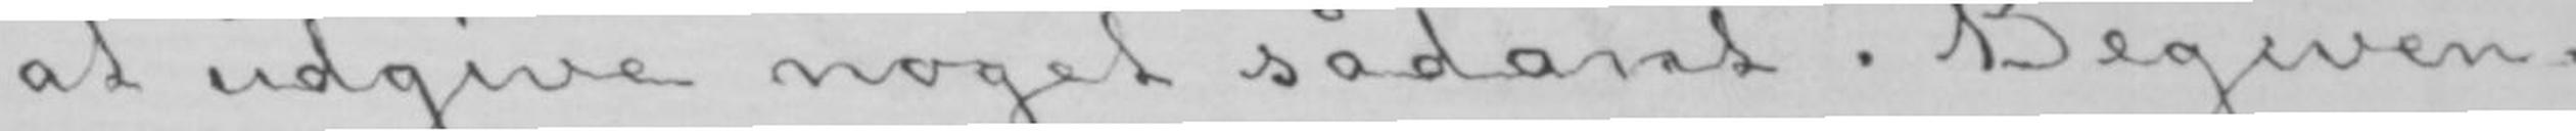at udgive noget sådant. Begiven-
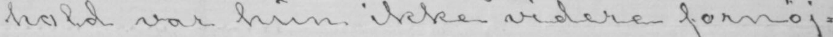hold var hun ikke videre fornøj-
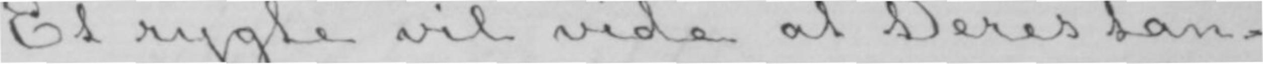Et rygte vil vide at Deres tan-
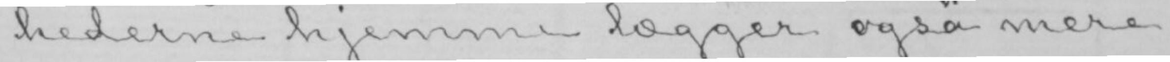hederne hjemme lægger også mere
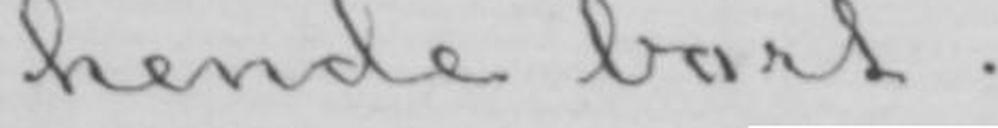hende bort.
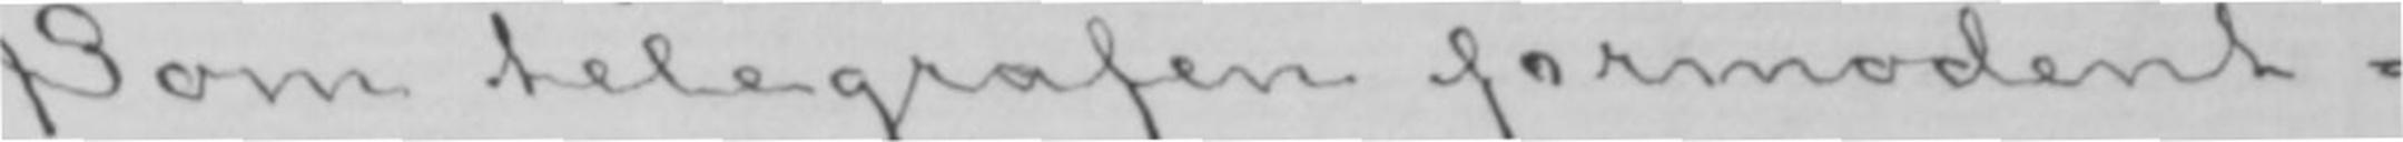Som telegrafen formodent-
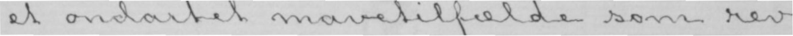et ondartet mavetilfælde som rev
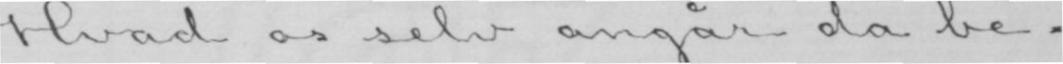Hvad os selv angår da be-
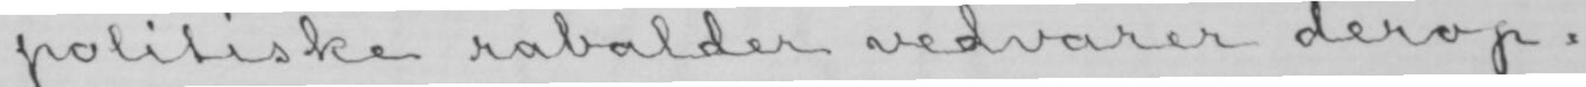politiske rabalder vedvarer derop-
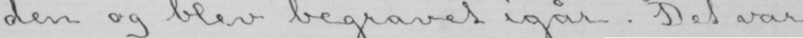den og blev begravet igår. Det var
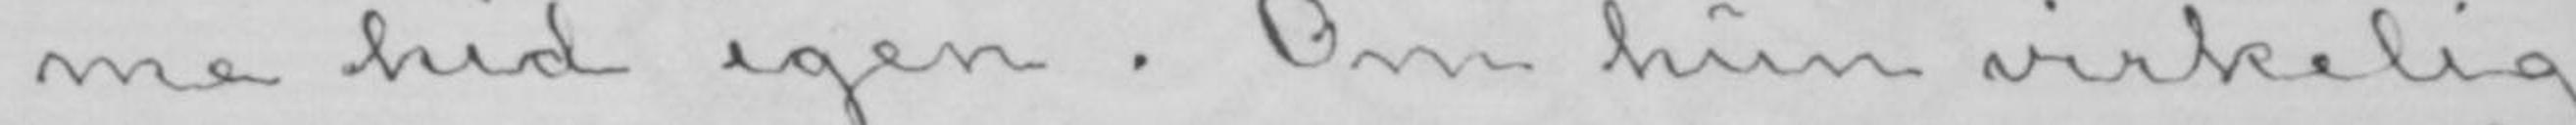me hid igen. Om hun virkelig
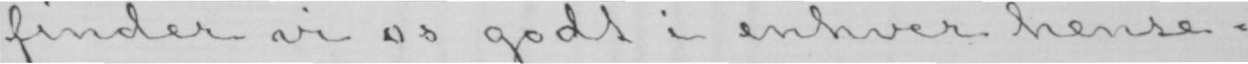finder vi os godt i enhver hense-
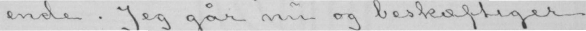ende. Jeg går nu og beskæftiger
